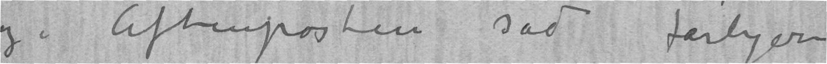og i Aftenposten sad farligere
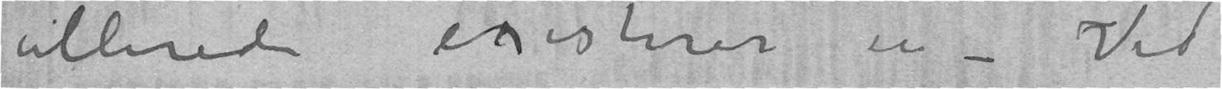allerede existerer en – Ved
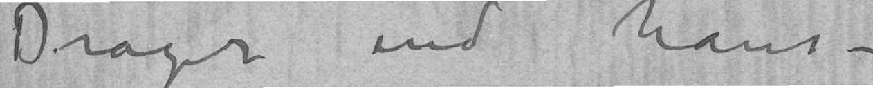Drager end hans –
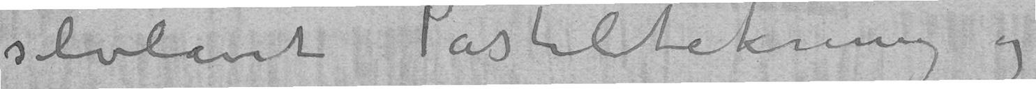selvlavet Pasteltekning og
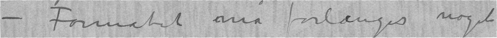–	Formatet må forlænges noget
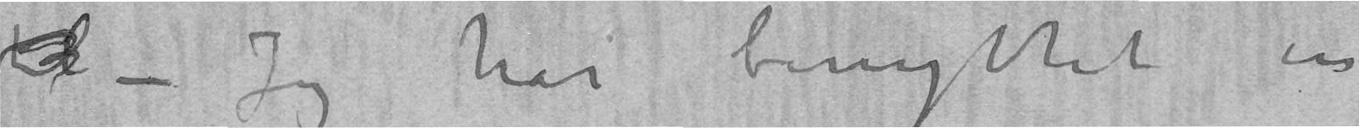til –	Jeg har benyttet en
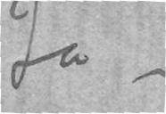få –
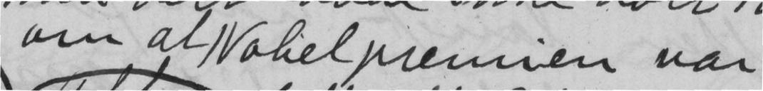om at Nobelpremien var
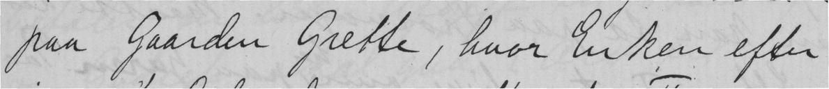paa Gaarden Grette, hvor Enken efter
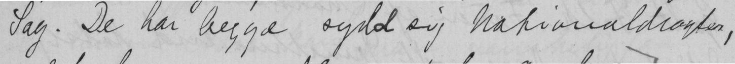Sag. De har begge sydd sig nationaldragter,
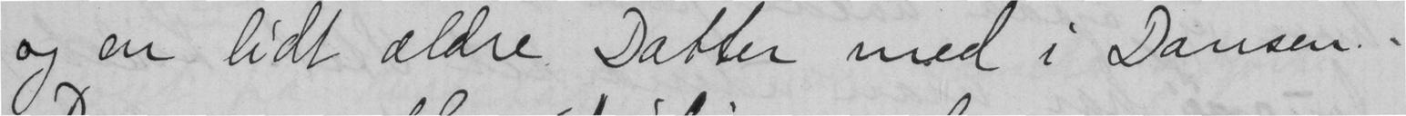og en lidt ældre Datter med i Dansen.
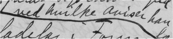med hvilke aviser han
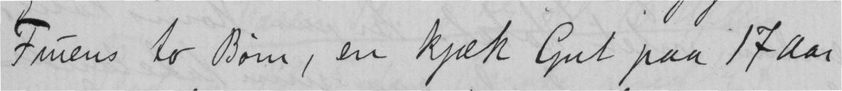Fruens to Børn, en kjæk Gut paa 17 Aar
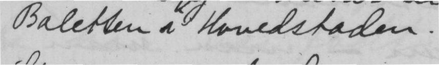Baletten i "Hovedstaden".
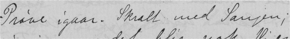Prøve igaar. Skralt med Sangen;
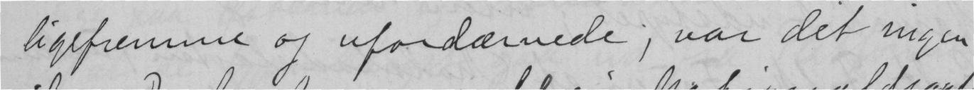ligefremme og ufordærvede, var det ingen
